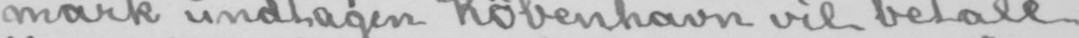mark undtagen København vil betale
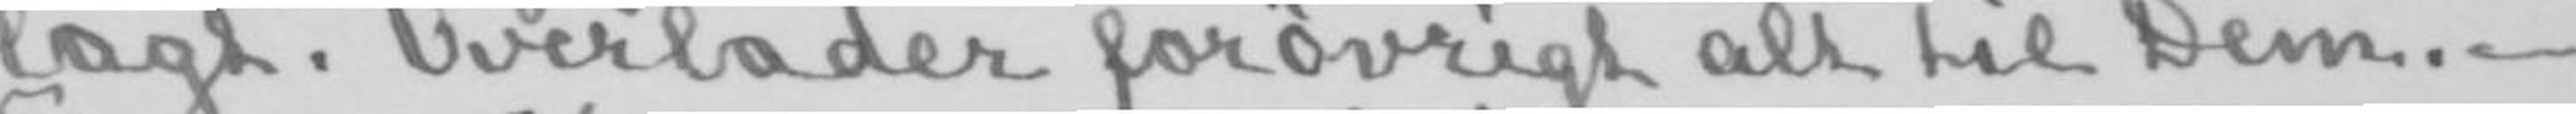lagt. Overlader forøvrigt alt til Dem.-
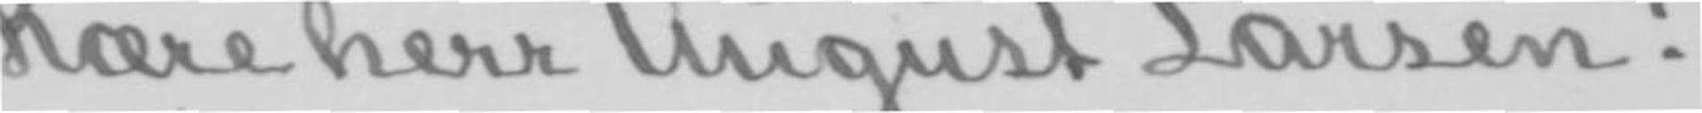Kære herr August Larsen!
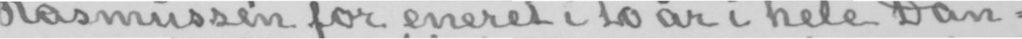Rasmussen for eneret i to år i hele Dan-
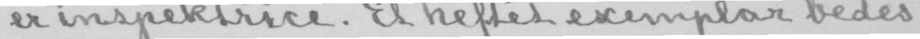er inspektrice. Et heftet exemplar bedes
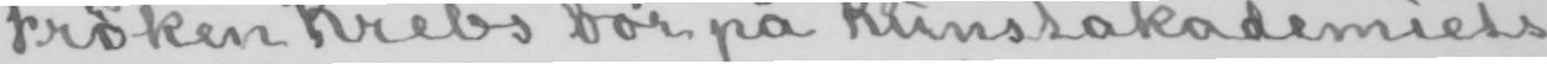Frøken Krebs bor på Kunstakademiets
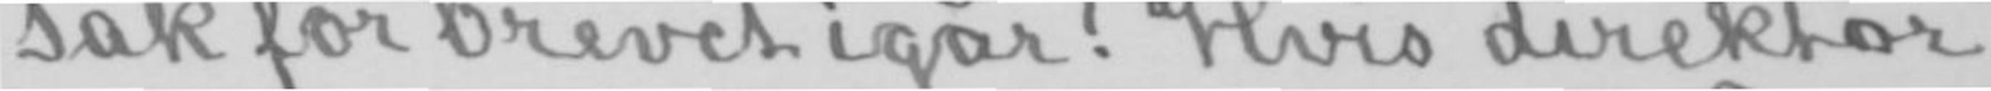Tak for brevet igår! Hvis direktør
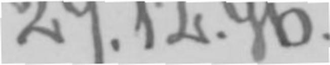29.12.96.
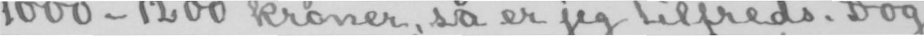1000-1200 kroner, så er jeg tilfreds. Dog
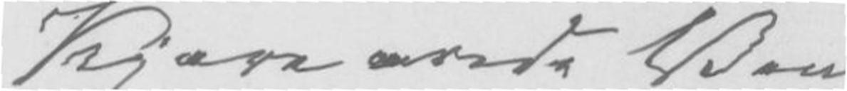Kjære ærede Ven
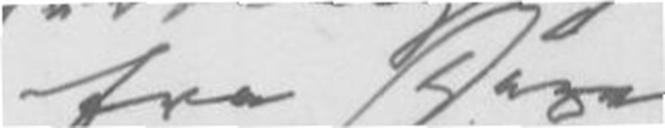fra Deres
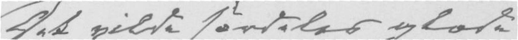Det vilde serdeles glæde
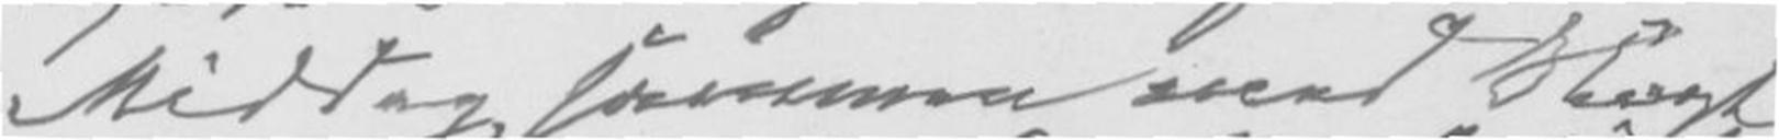Middag sammen med Slægt
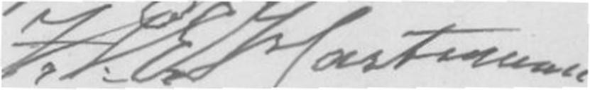J.P.E.Hartmann
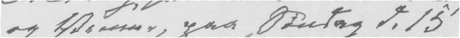og Venner, paa Søndag d. 15
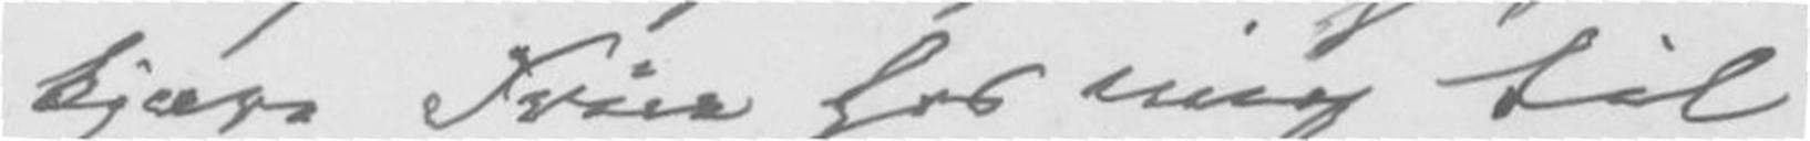kjære Frue hos mig til
